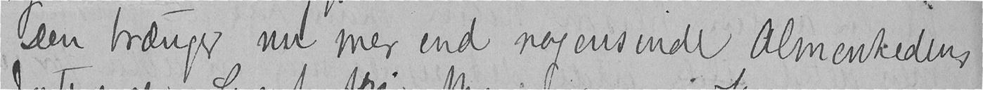Den trænger nu mer end nogensinde Almenhedens
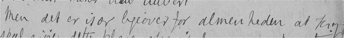Men det er især ligeover for almenheden, at Krogh
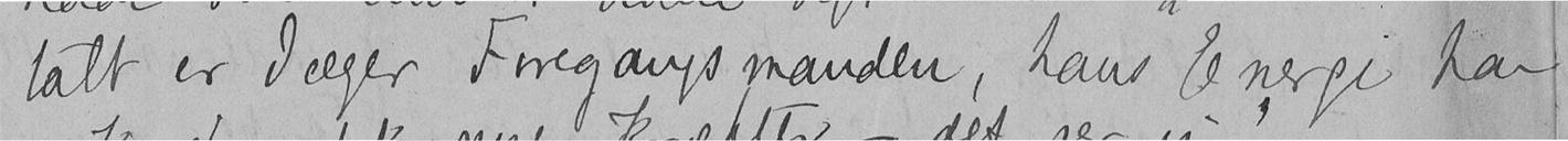talt er Jæger Foregangsmanden, hans Energi har
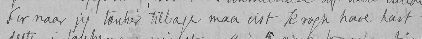For naar jeg tænker tilbage maa vist Krogh have havt
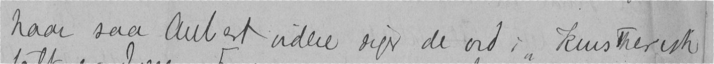Naar saa Aubert videre siger de ord: "kunstnerisk
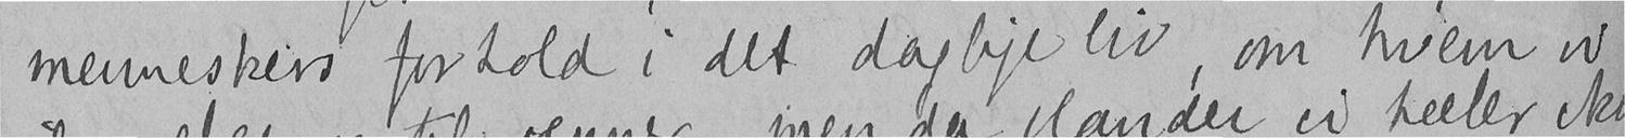menneskers forhold i det daglige liv, om hvem vi
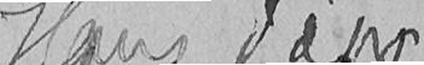Hans Jæger
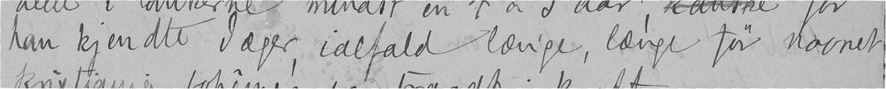han kjendte Jæger, ialfald længe, længe før navnet
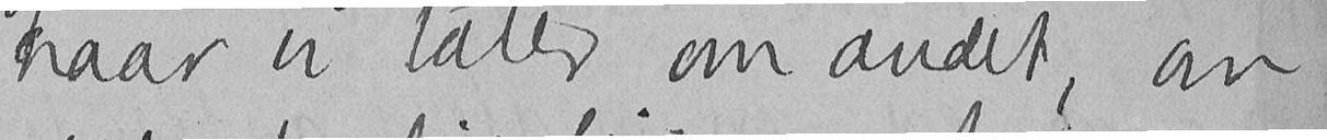naar vi taler om andet, om
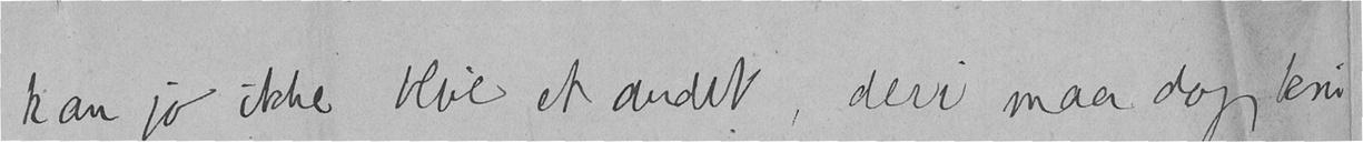kan jo ikke blive et andet, deri maa dog kri
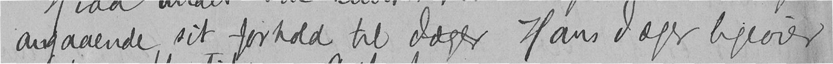angaaende sit forhold til Jæger Hans Jæger ligeover
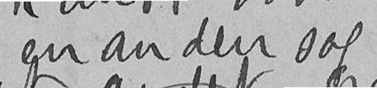en anden sag
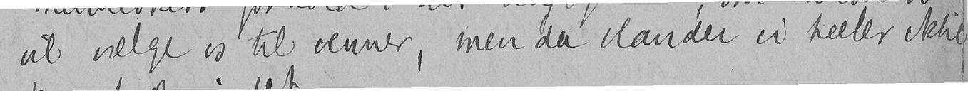vil vælge os til venner, men da blander vi heller ikke
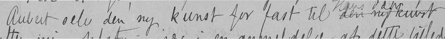Aubert selv den ny kunst for fast til den ny kunst
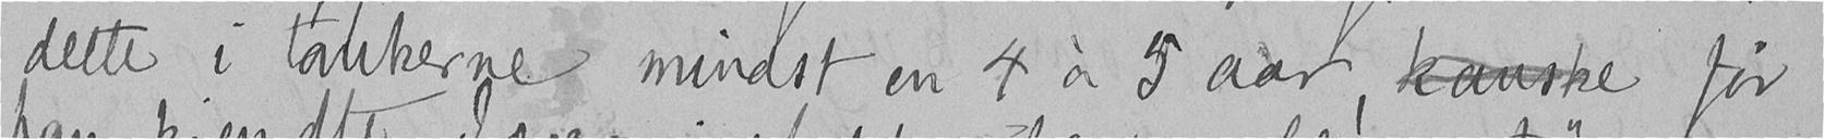dette i tankerne mindst en 4 à 5 aar, kanske før
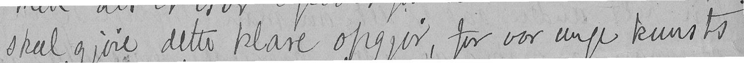skal gjøre dette klare opgjør, for vor unge kunsts
